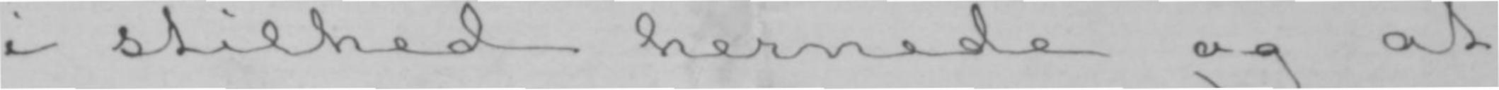i stilhed hernede og at
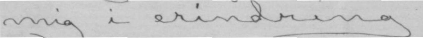mig i erindring.
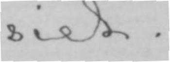siet.
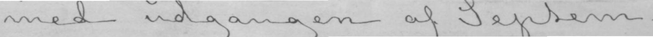med udgangen af Septem-
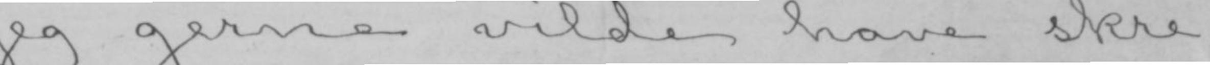jeg gerne vilde have skre-
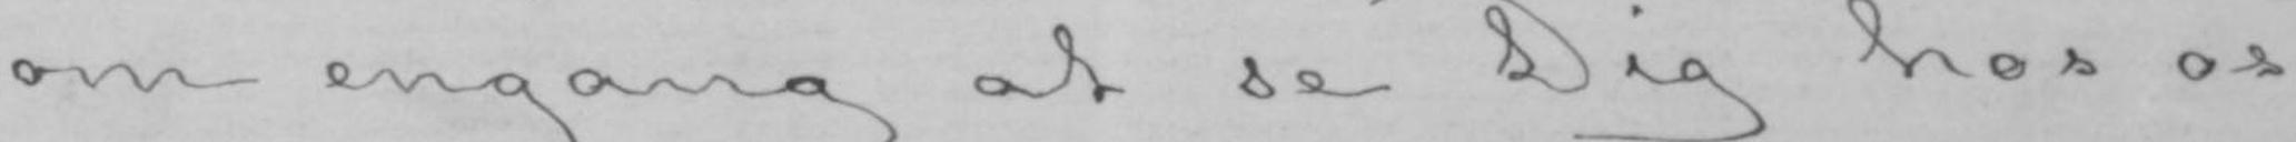om engang at se Dig hos os
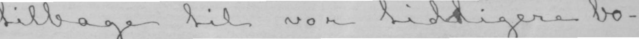tilbage til vor tidligere bo-
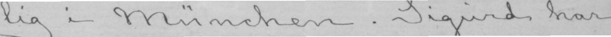lig i München. Sigurd har
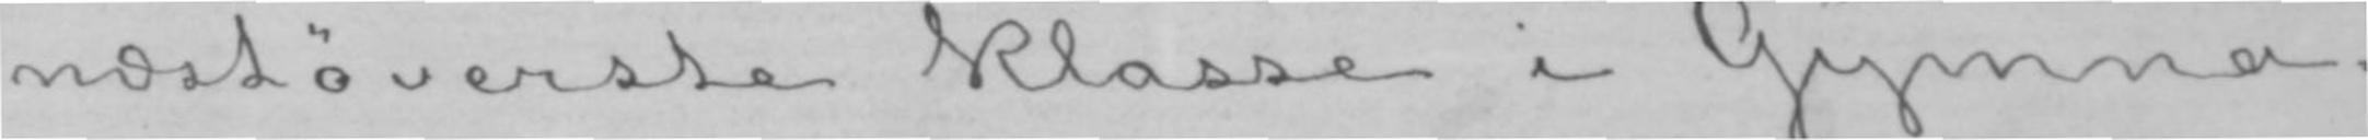næstøverste klasse i Gymna-
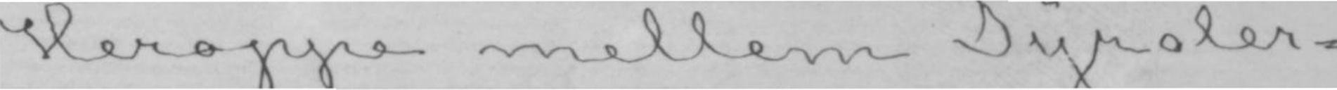Heroppe mellem Tyroler-
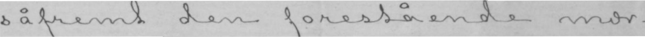såfremt den forestående mær-
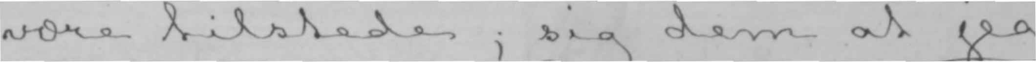være tilstede; sig dem at jeg
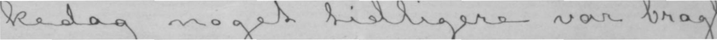kedag noget tidligere var bragt
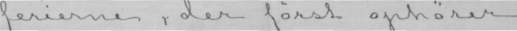ferierne, der først ophører
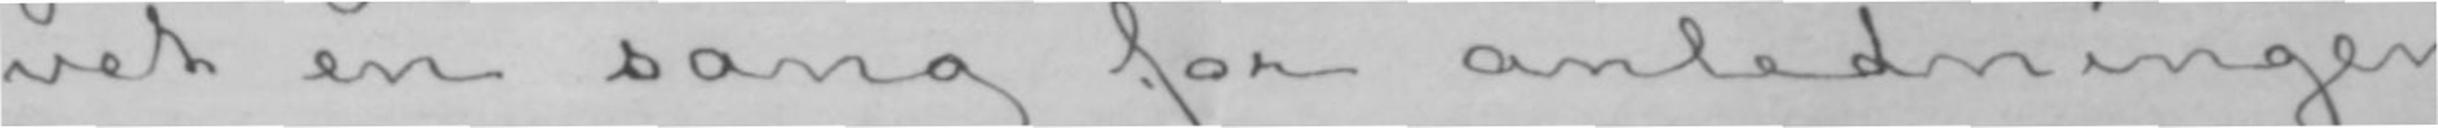vet en sang for anledningen,
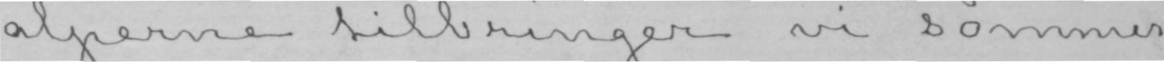alperne tilbringer vi sommer-
